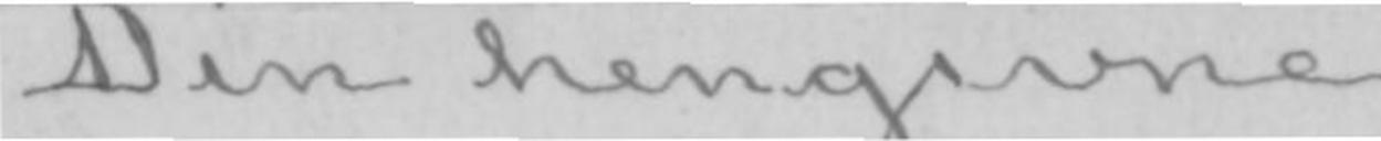Din hengivne
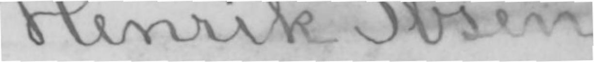Henrik Ibsen.
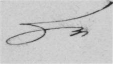Hr
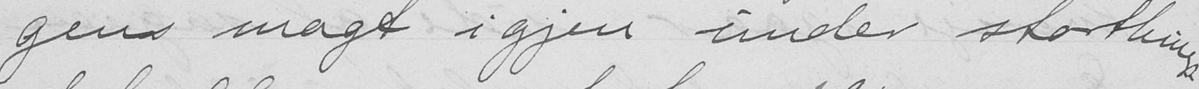gens magt igjen under storthings-
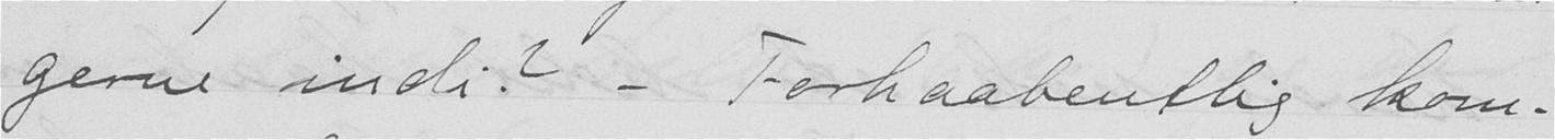gerne indi? - Forhaabentlig kom-
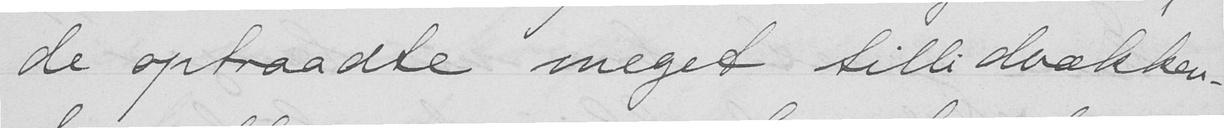de optraadte meget tillidvækken-
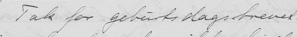Tak for geburtsdagsbrevet!
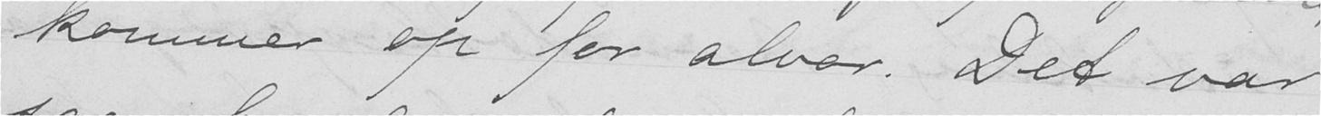kommer op for alvor. Det var
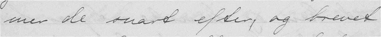mer de snart efter, og brevet
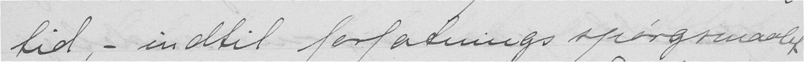tid, - indtil forfatningsspørgsmaalet
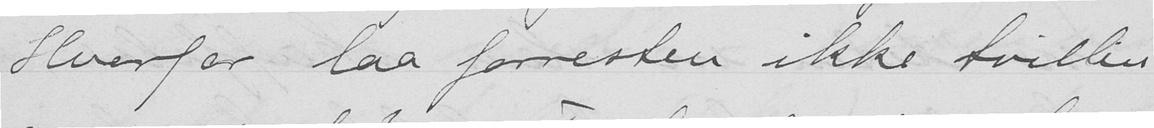Hvorfor laa forresten ikke tvillin-
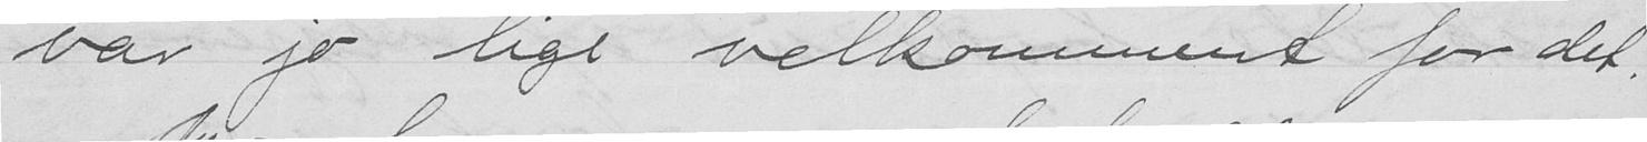var jo lige velkomment for det.
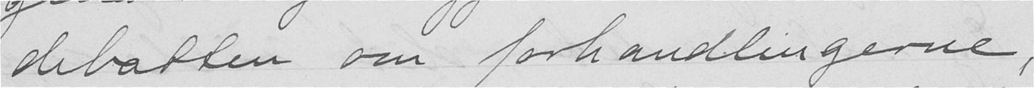debatten om forhandlingerne;
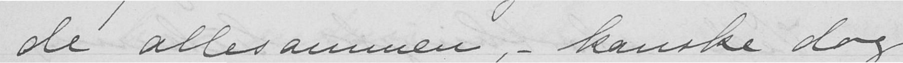de allesammen, - kanske dog
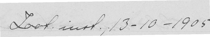Zoot. inst., 13-10-1905
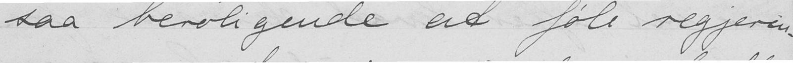saa beroligende at føle regjerin-
